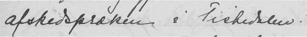afskedspræken i Tistedalen.
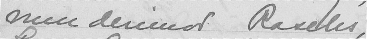men derimod Raschs,
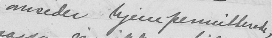omsider "hjempermitterede",
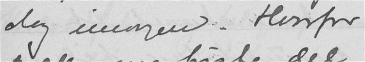dag imorgen. Hvorfor
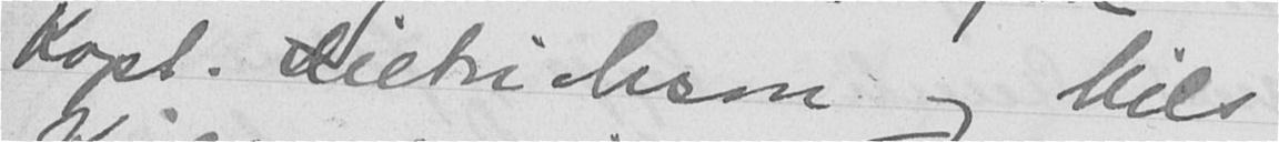Kapt. Dietrichson og Nils
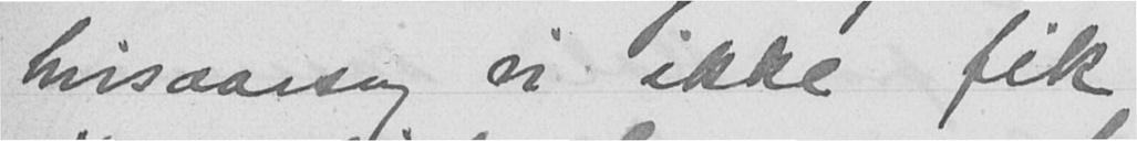hvisaarsag vi ikke fik
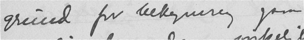grund for bekymring ogsaa
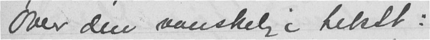Over den vanskelige tekst:
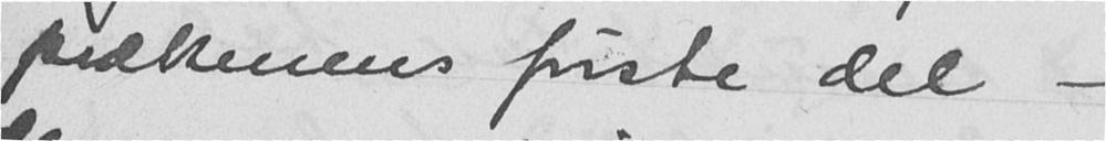prækenens første del -
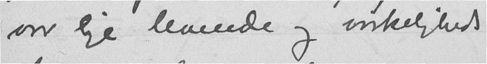var lige levende og virkeligheds
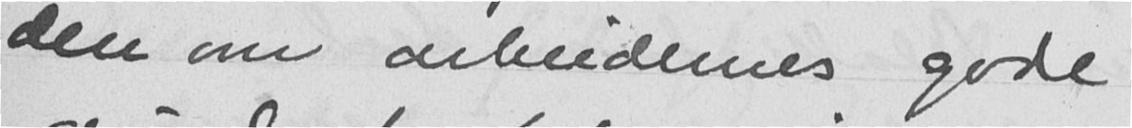den om arbeidernes gode
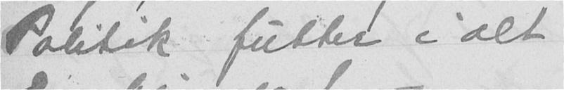Politik futter i alt
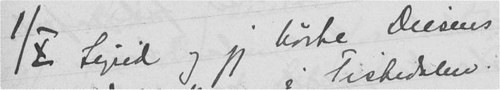1/X Sigrid og jeg hørte Diesens
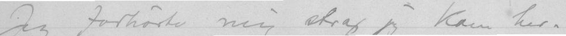Jeg forhørte mig stax jeg kom her-
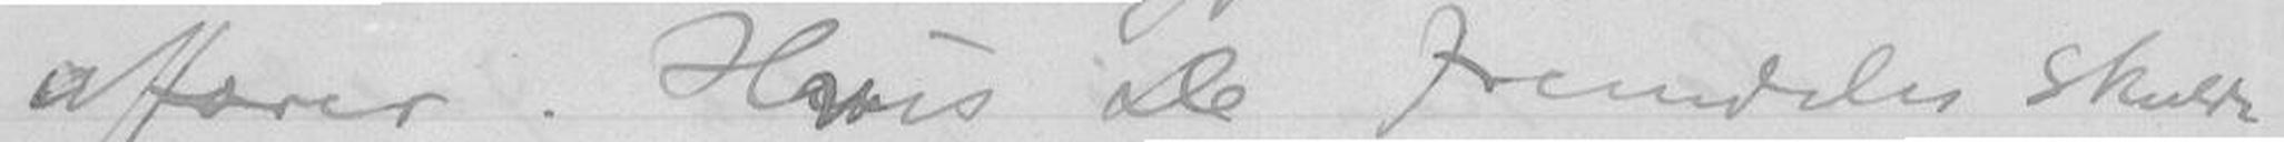affærer. Hvis De fremdeles skulde
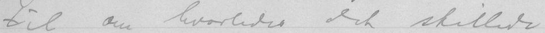til om hvorledes det stillede
